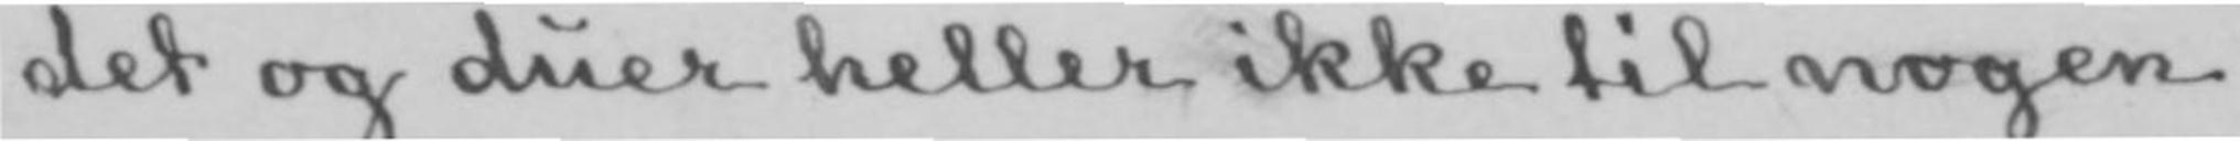det og duer heller ikke til nogen
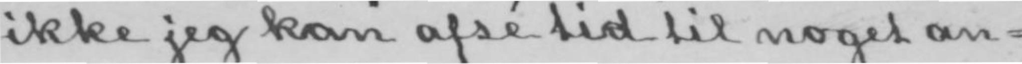ikke jeg kan afsé tid til noget an-
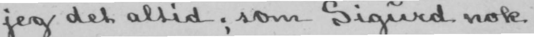jeg det altid; som Sigurd nok
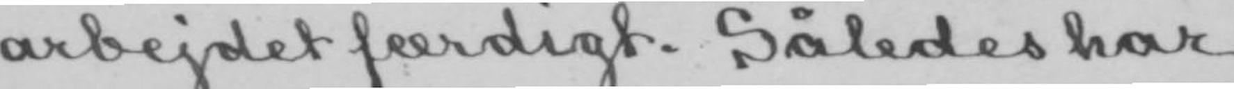arbejdet færdigt. Således har
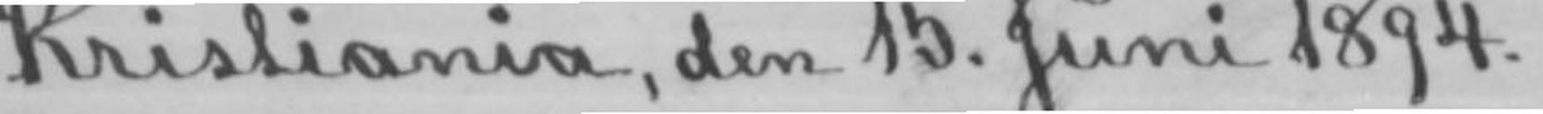Kristiania, den 15. Juni 1894.
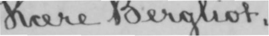Kære Bergliot,
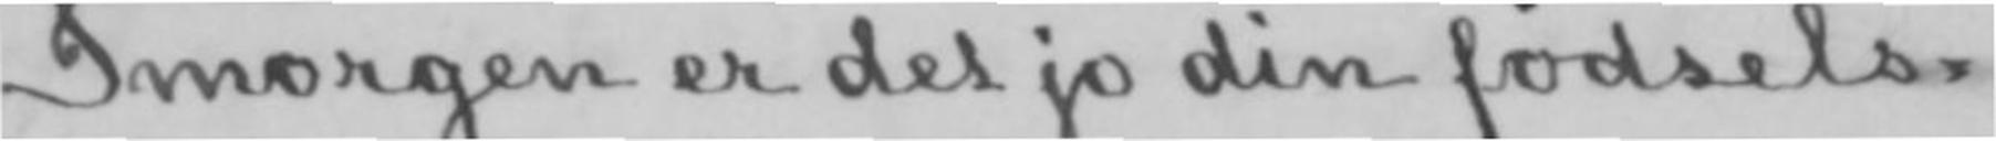Imorgen er det jo din fødsels-
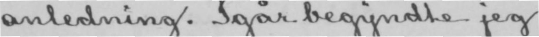anledning. Igår begyndte jeg
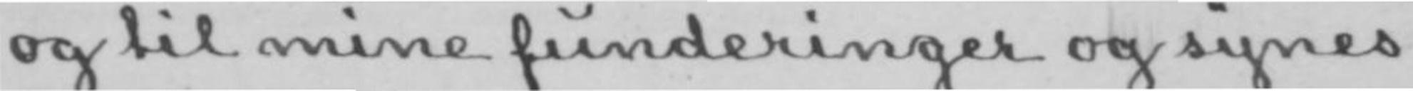og til mine funderinger og synes
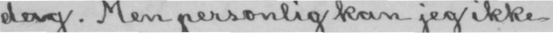deag. Men personlig kan jeg ikke
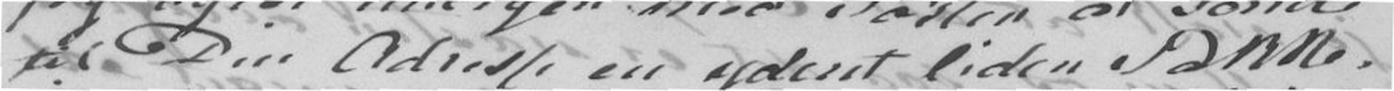til Din Adresse en yderst liden Pakke,
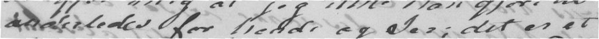anderledes for hende og Jer; det er et
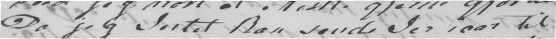Da jeg Intet kan sende Jer iaar til
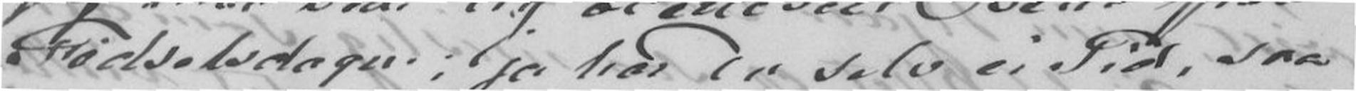Fødselsdagen; ja har Du selv ei Tid, saa
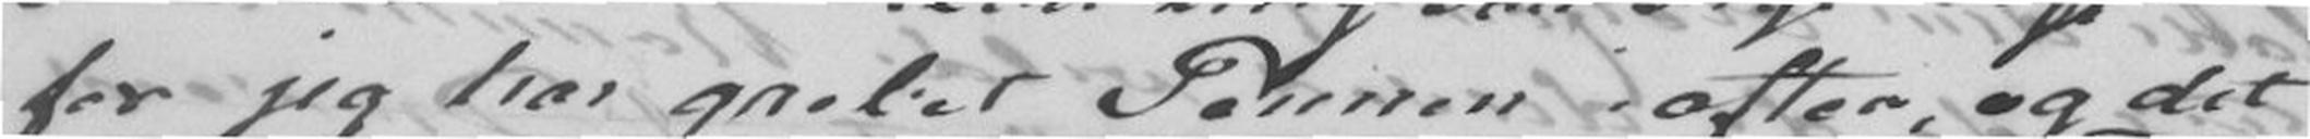for jeg har grebet Pennen iaften, og det
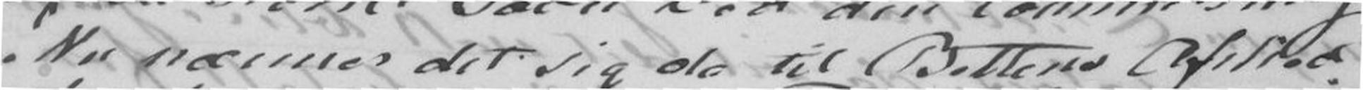Nu nærmer det sig da til Bettens Afsked
